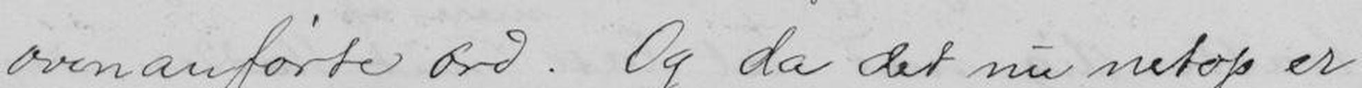ovenanførte Ord. Og da det nu netop er
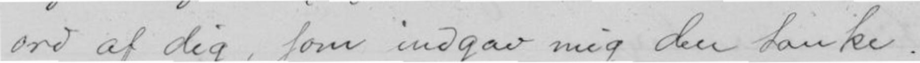ord af dig, som indgav mig den tanke.
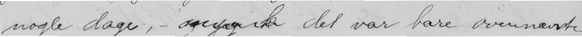nogle dage, - det var bare ovennemte
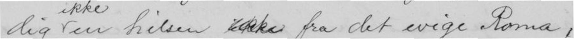dig en hilsen fra det evige, Roma;
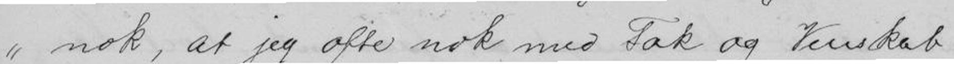"nok, at jeg ofte nok med Tak og Venskab
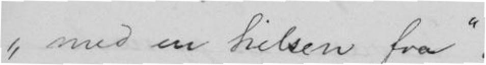"med en hilsen fra "
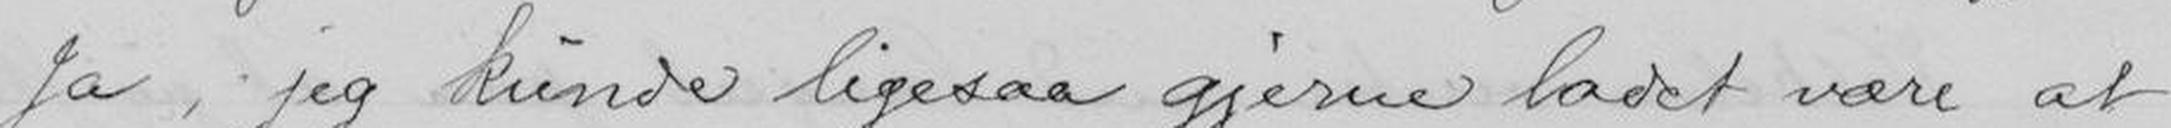Ja, jeg kunde ligesaa gjerne ladet være at
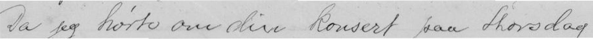Da jeg hørte om din konsert paa thorsdag
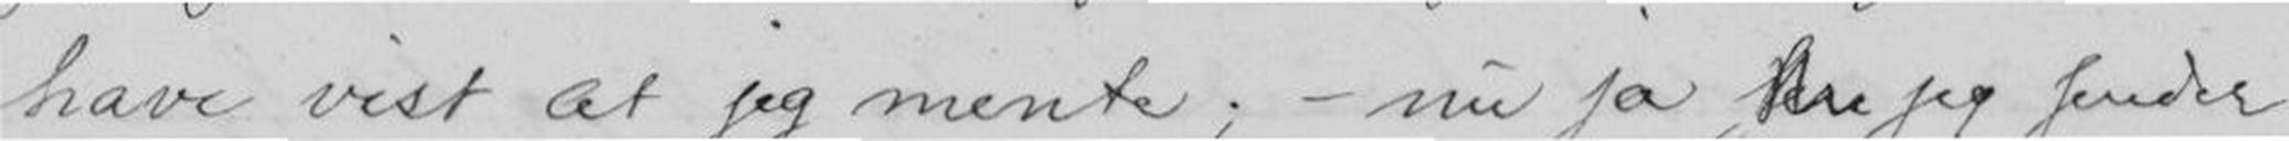have vist at jeg mente; - nu ja jeg sender
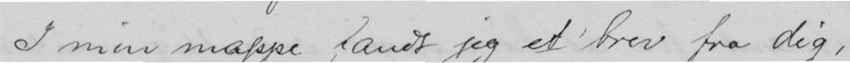I min mappe fandt jeg brev fra dig,
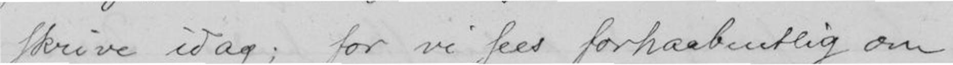skrive idag; for vi sees forhaabentlig om
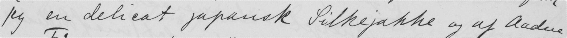jeg en delicat japansk Silkejakke og af Aadne
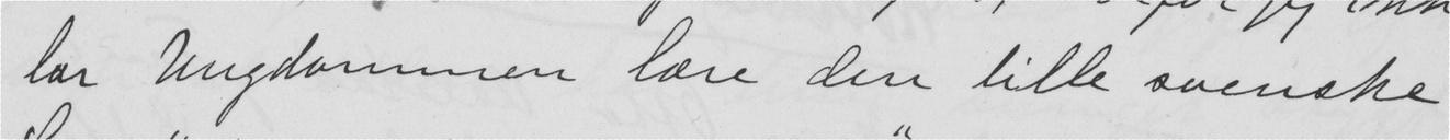lar Ungdommen lære den lille svenske
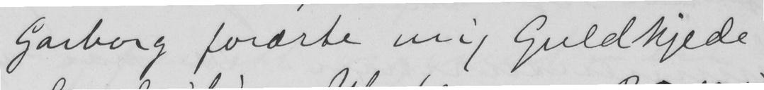Garborg forærte mig Guldkjede
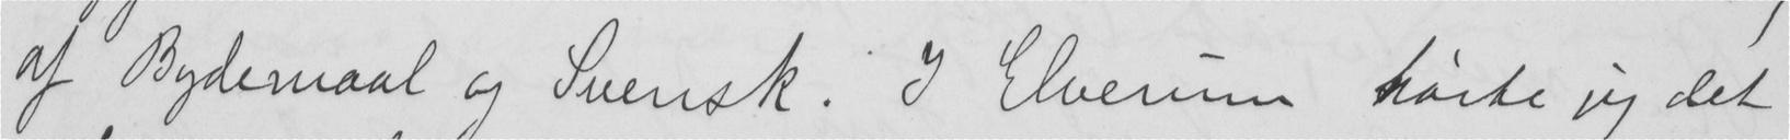af Bydemaal og Svensk. I Elverum hørte jeg det
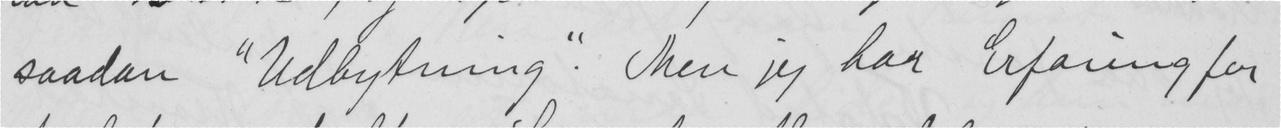saadan. "Udbytning". Men jeg har Erfarig for
